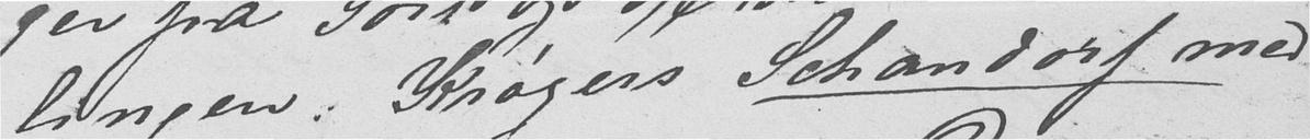lingen. Krøyers Schandorf med
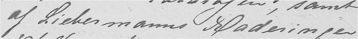af Liebermanns Raderinger
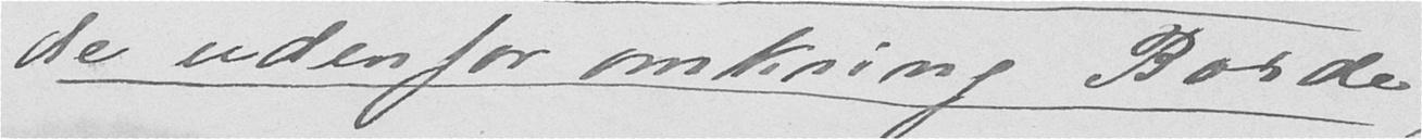de udenfor omkring Borde,
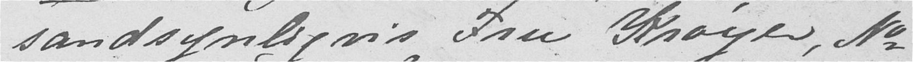sandsynligvis Fru Krøyer. No
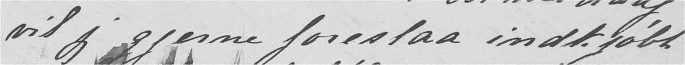vil jeg gjerne foreslaa indkjøbt
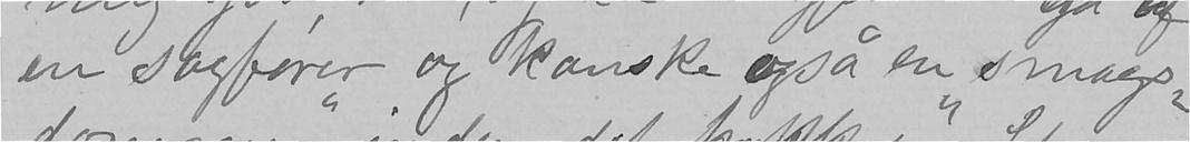en sagfører og kanske også en "smags-
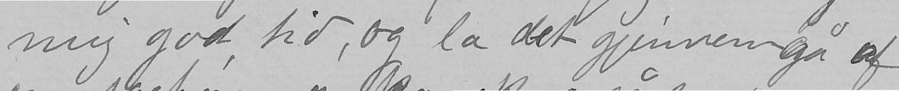mig god tid, og la det gjennemgå af
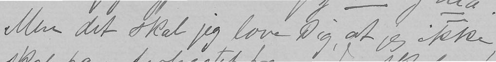Men det skal jeg love Dig, at jeg ikke
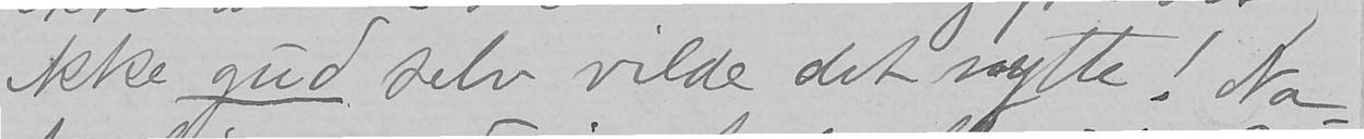ikke gud selv vilde det nytte! Na-
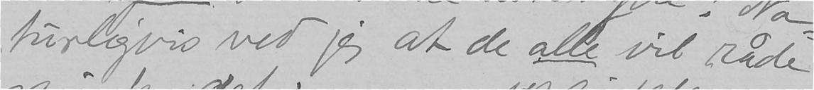turligvis ved jeg at de alle vil råde
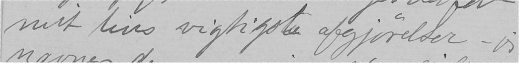mit livs vigtigste afgjørelser - jeg
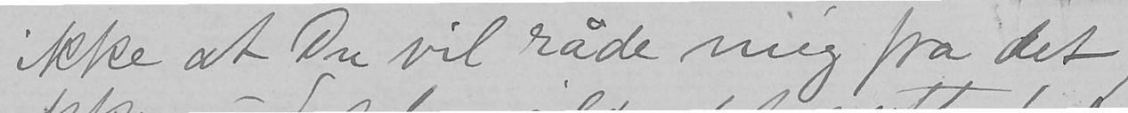ikke at Du vil råde mig fra det
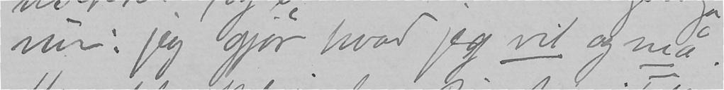nu: jeg gjør hvad jeg vil og må.
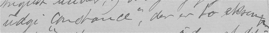udgi "Constance", der er to eksemp-
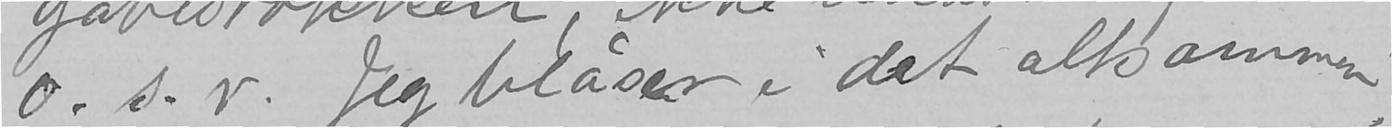o. s.v. Jeg blåser i det altsammen.
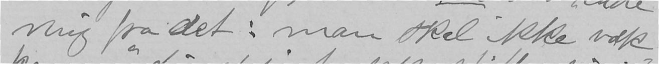mig fra det: man skal ikke væk-
